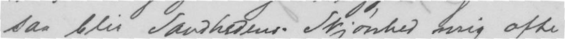saa blir Sandhedens Skjønhed mig ofte
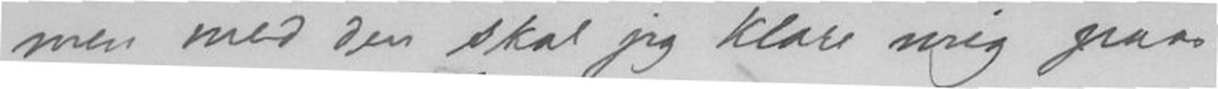men med den skal jeg klare mig paa
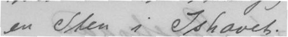en Sten i Ishavet.
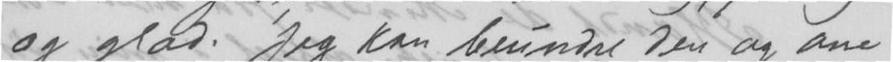og glad. Jeg kan beundre den og ane
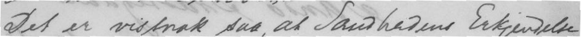Det er vistnok saa, at Sandhedens Erkjendelse
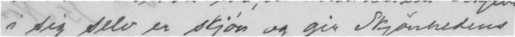i sig selv er skjøn og gir Skjønhedens
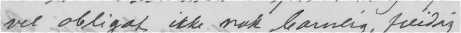vel obligat, ikke nok barnlig, freidig
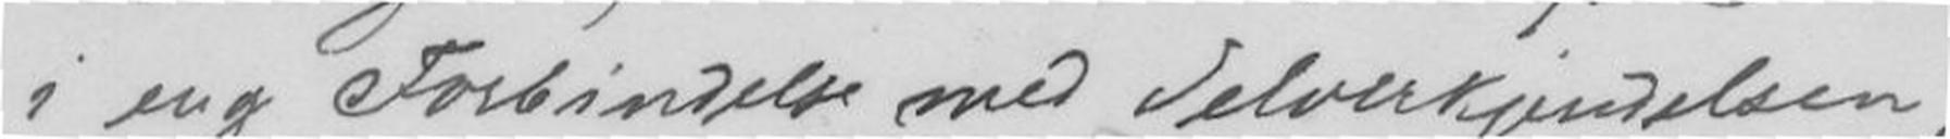i eng Forbindelse med Selverkjendelsen,
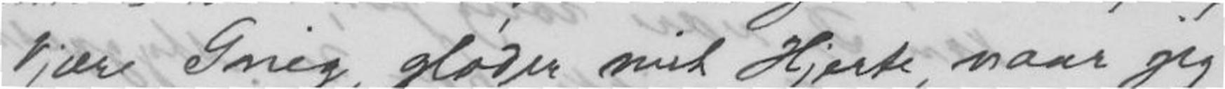kjære Grieg, glæder mit Hjerte, naar jeg
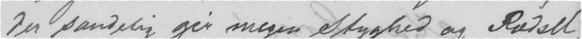der sandelig gir megen Styghed og Rædsel,
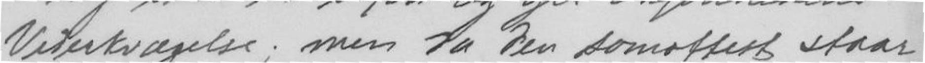Vederkvægelse; men da den som oftest staar
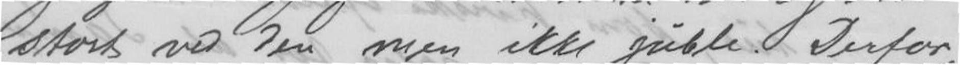stort ved den men ikke juble. Derfor,
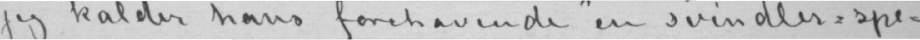jeg kalder hans forehavende «en svindler-spe-
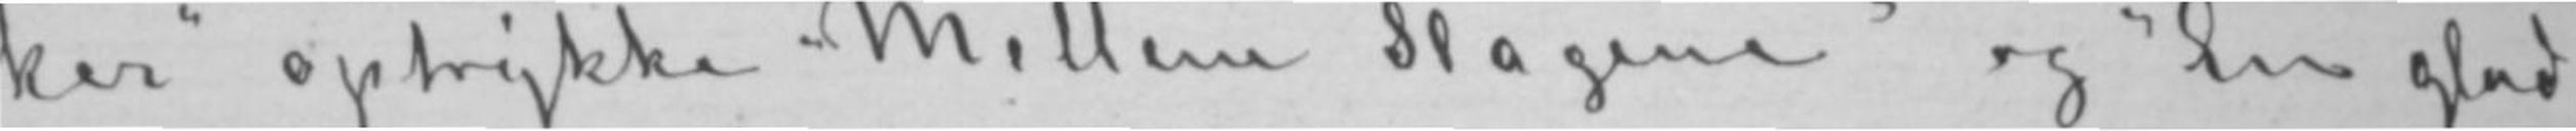ker» optrykke «Mellem Slagene» og «En glad
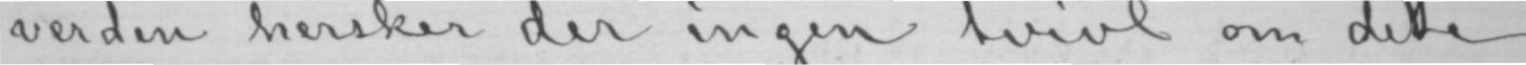verden hersker der ingen tvivl om dette
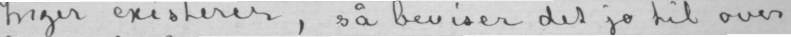Inger» existerer, så beviser det jo til over-
